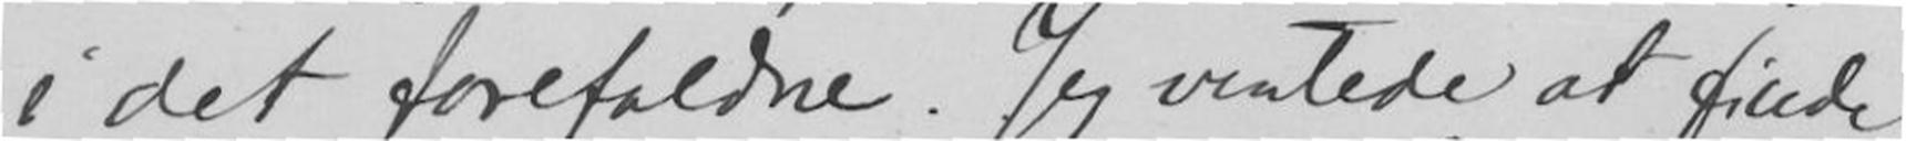i det forefaldne. jeg ventede at finde
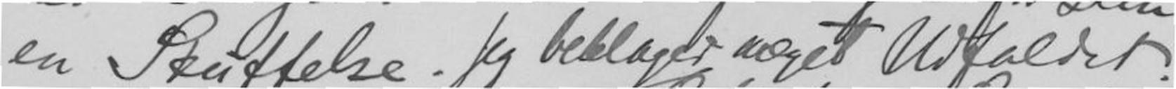en Skuffelse. Jeg beklager mæget Udfaldet.
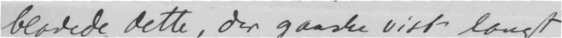bladede dette, derganske vist langt
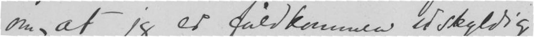om, at jeg er fuldkommen Uskyldig
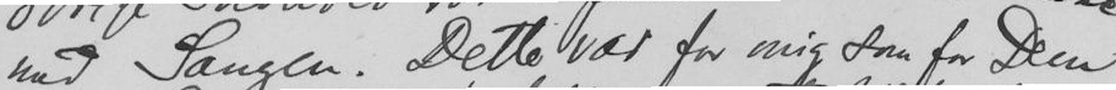med Sangen. Dette var for mig som for Dem
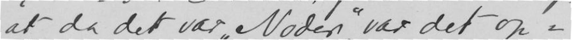at da det var "Noder", var det op-
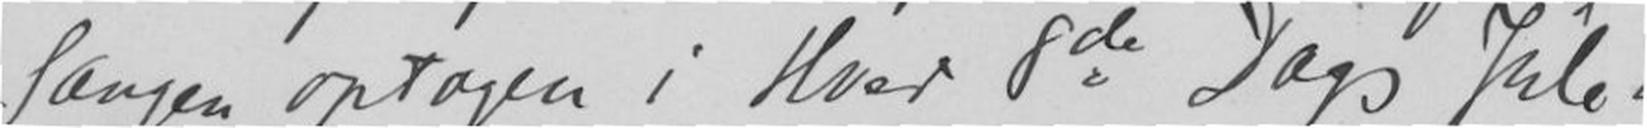Sangen optagen i 8de Dags Jule
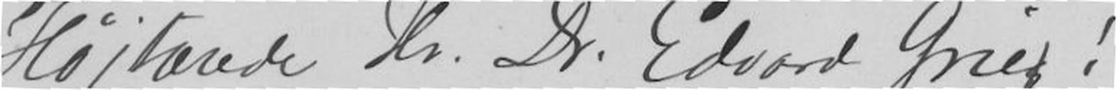Højtærede Hr. Dr. Edvard Grieg!
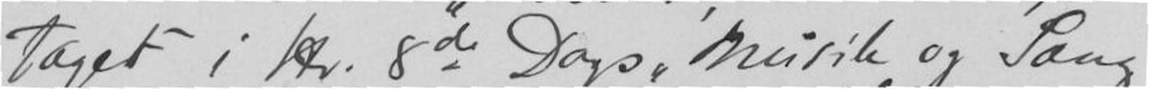taget i Den 8de Dags"Musik og Sang
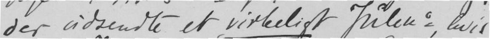der udsendte et virkeligt Juleno,
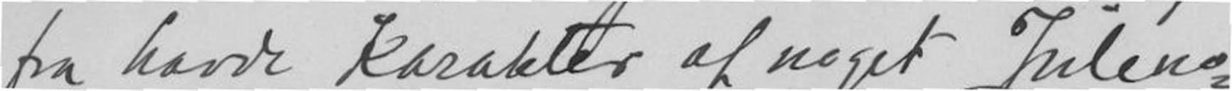fra havde Karakter af noget Juleno
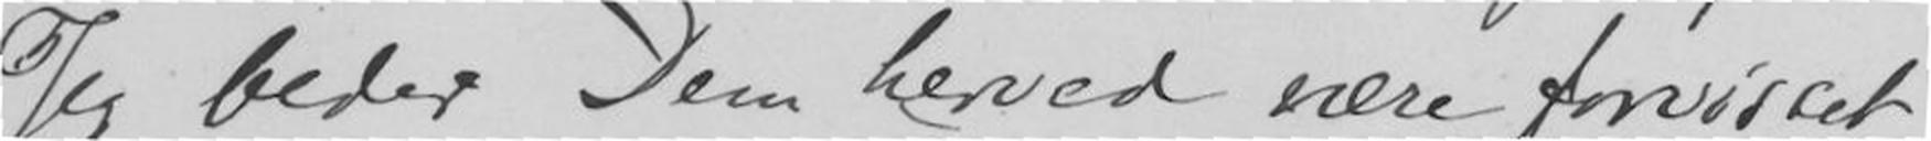Jeg beder Dem herved være forvisset
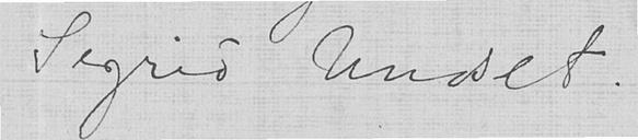Sigrid Undset.
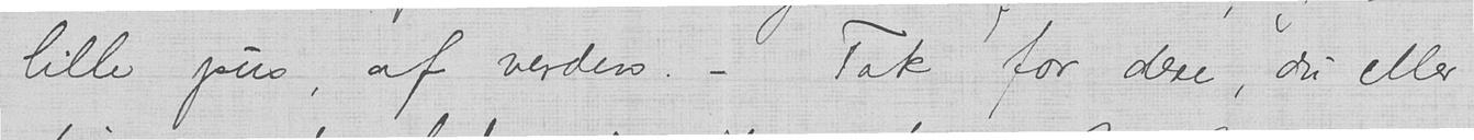lille pus, af verden. - Tak for dere, du eller
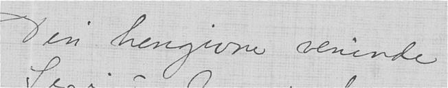Din hengivne veninde
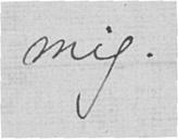mig.
